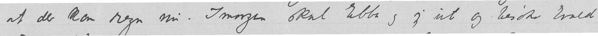at der kom regn nu.  Imorgen skal Ebba og jeg ut og besøke Evald
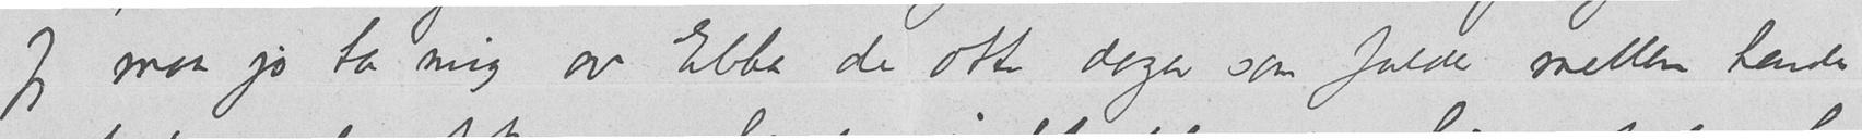Jeg maa jo ta mig av Ebba de otte dager som falder mellem hendes
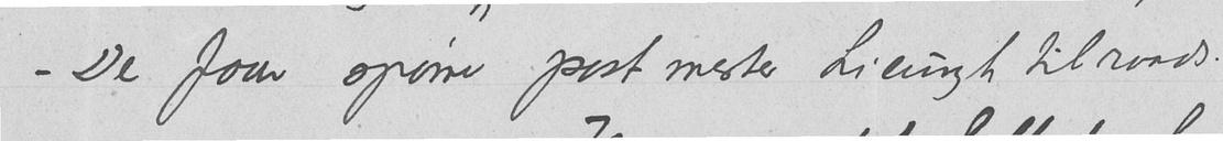– De faar spørre postmester Lieungh tilraads.
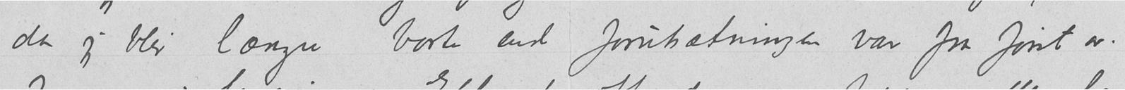da jeg blir længre borte end forutsætningen var fra først av.
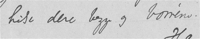hilse dere begge og børnene.
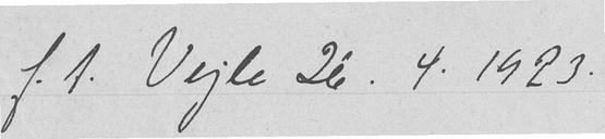f.t. Vejle 26.4.1923.
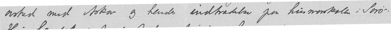avsked med Askov og hendes indtrædelse paa husmorskolen i Sorø –.
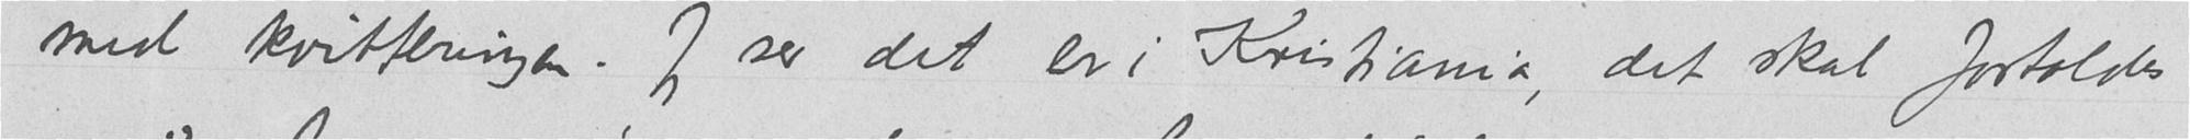med kvitteringen. Jeg ser det er i Kristiania, det skal fortoldes
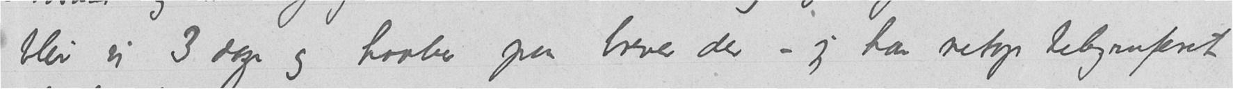blir vi 3 dager og haaber paa brever der – jeg har netop telegraferet
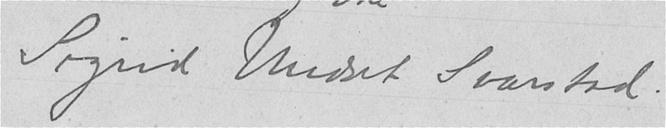Sigrid Undset Svarstad.
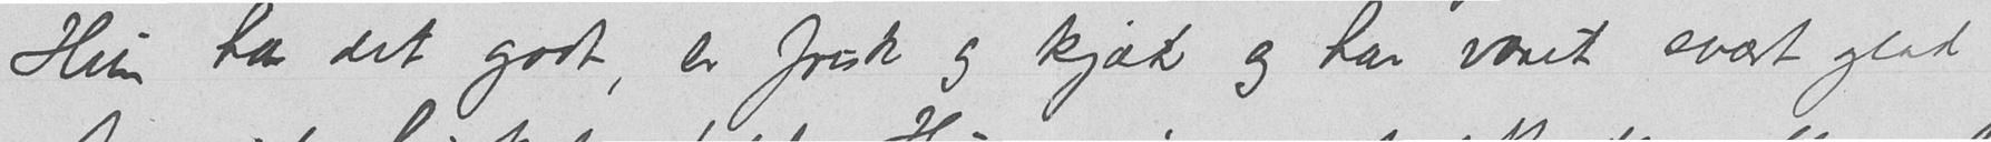Hun har det godt, er frisk og kjæk og har været svært glad
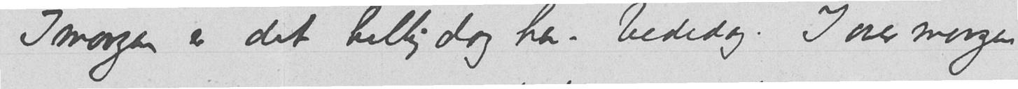Imorgen er det helligdag her – bededag. I overmorgen
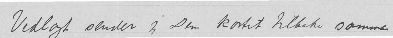Vedlagt sender jeg Dem kortet tilbake sammen
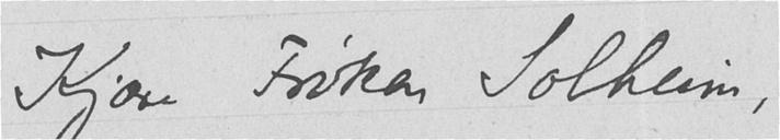Kjære Frøken Solheim,
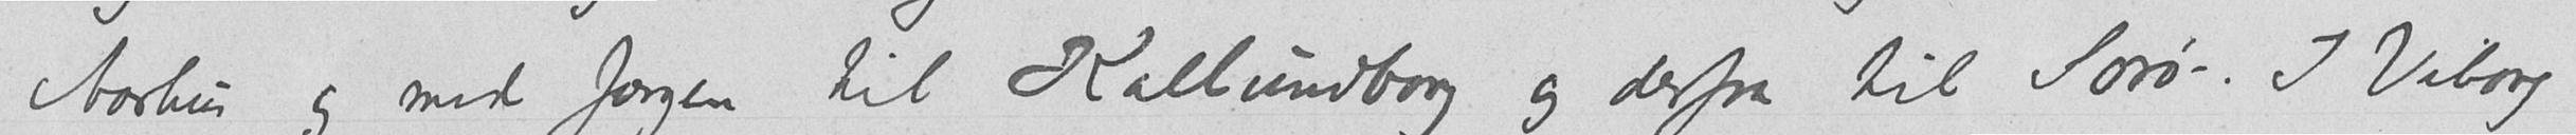Aarhus og med færgen til Kallundborg og derfra til Sorø – . I Viborg
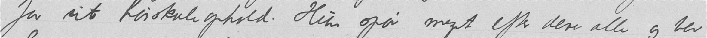for sit høiskoleophold. Hun spør meget efter dere alle og ber
